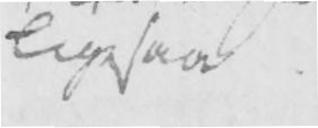ligesaa.
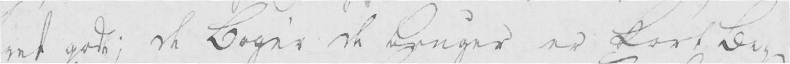ret godt; de Bøger de bruger er kort Be-
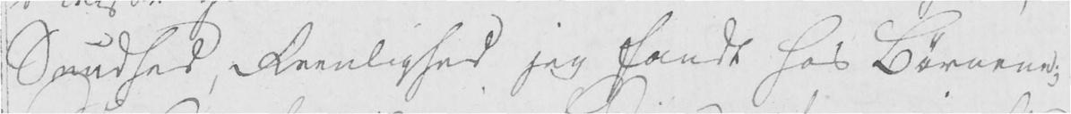Sundhed, Reenlighed jeg fandt hos Børnene;
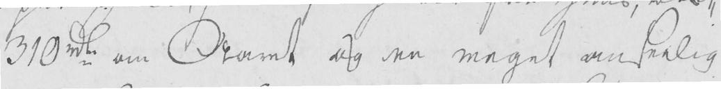310 rde om Aaaret og en meget anseelig
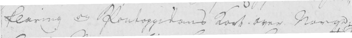klaring og Pontoppidans Kart over Norge.
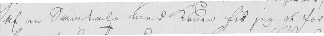af en Samtale med Konen fik jeg de for
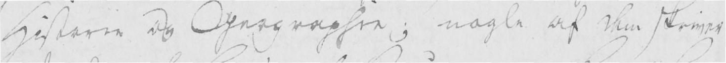Historie og Geographie; nogle af dem skriver
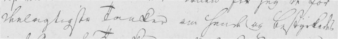deelagtigste Tanker om hende og bestyrkedes
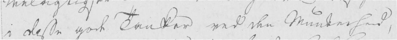i disse gode Tanker ved den Munterhed,
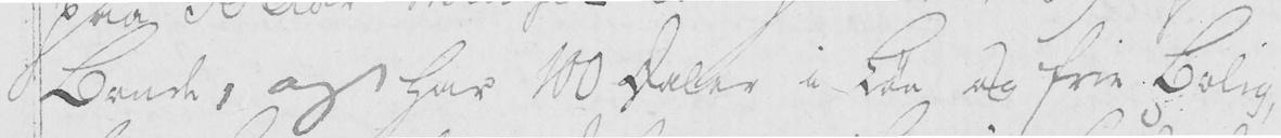Bonde, og har 100 Daler i Løn og frie Bolig,
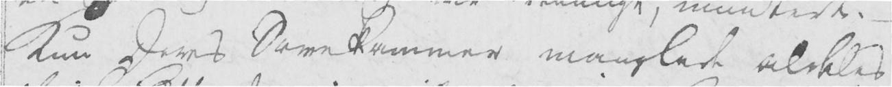kun Deres Sovekammer manglede aldeles
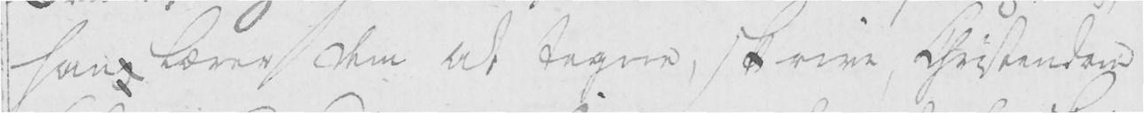han lærer dem at tegne, skrive, Christendom
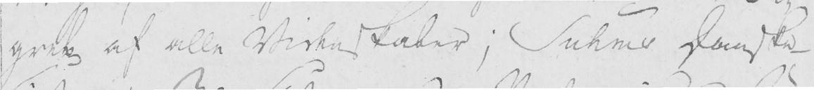greb af alle Videnskaber; Suhms Danske
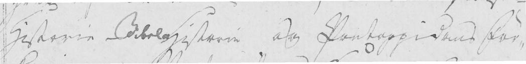Historie - Bibel-Historie og Pontoppidans For-
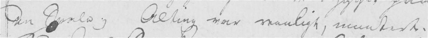en Svale; Alting var reenligt, muntert,
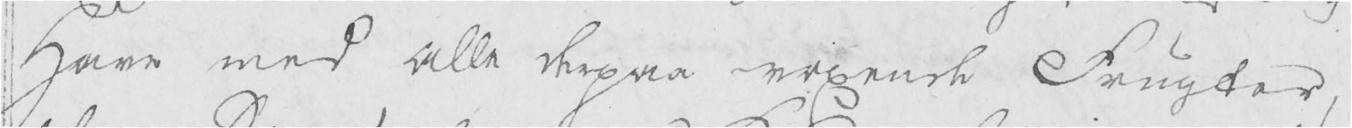Have med alle derpaa voxende Frugter,
